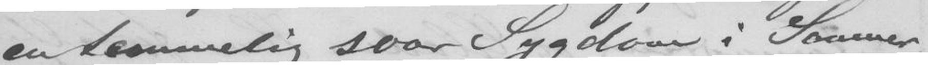en temmelig svor Sygdom i Sommer,
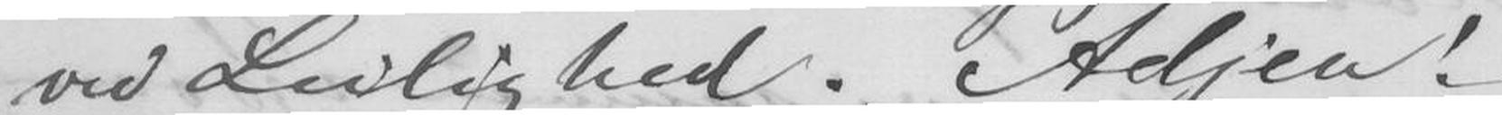ved Leilighed. Adjeu!
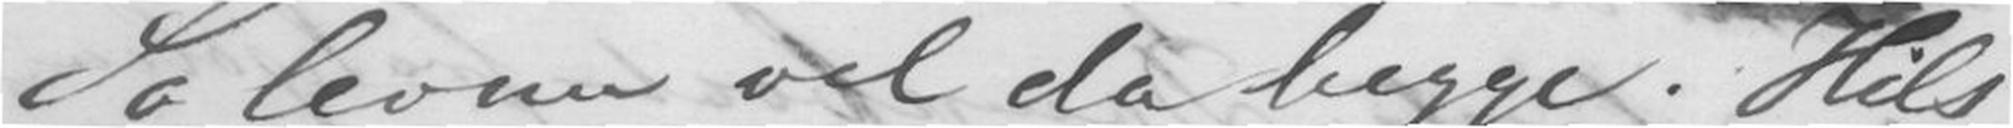So lev nu vel da begge. Hils
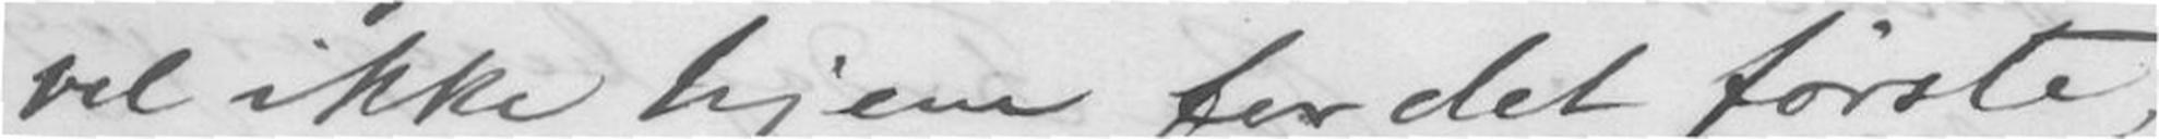vel ikke hjem for det første
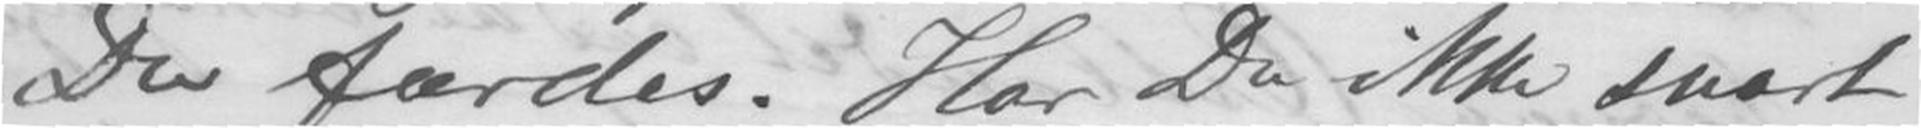Du færdes. Har Du ikke snart
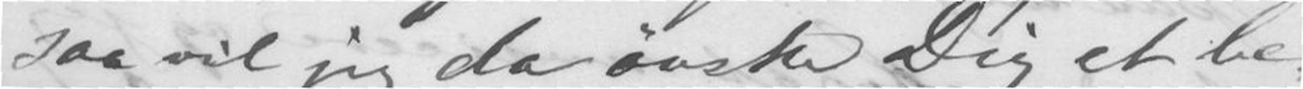saa vil jeg da ønske Dig et be-
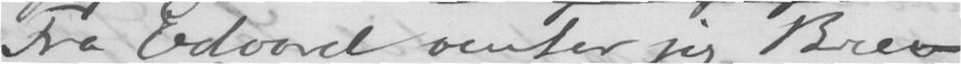Fra Edvard venter jeg Brev
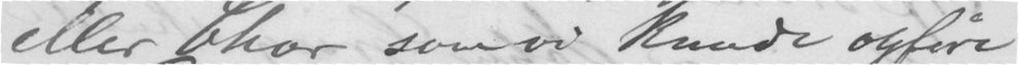eller Chor som vi kunde apføre
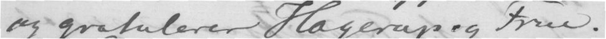og gratulerer Hagerup og Frue.
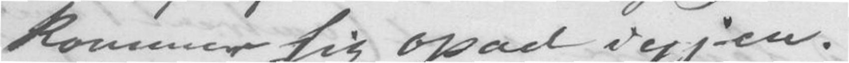kommer sig opad igjen.
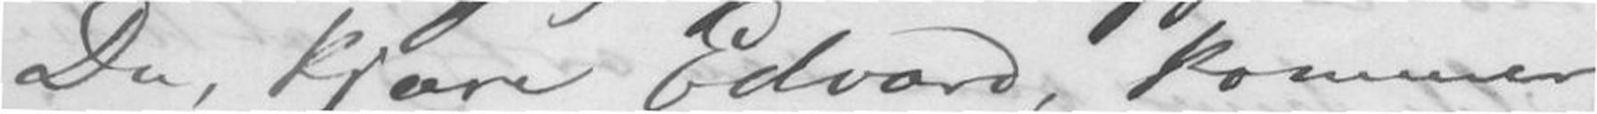Du, kjære Edvard, kommer
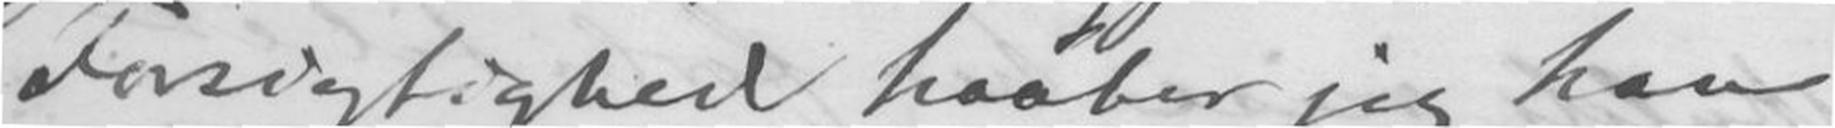Forsigtighed haaber jeg han
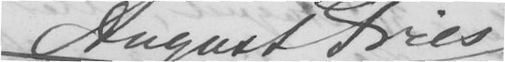August Fries
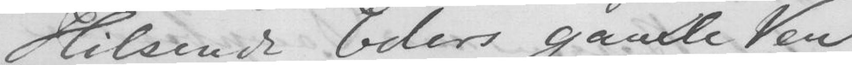Hilsende Ederes gamle Ven
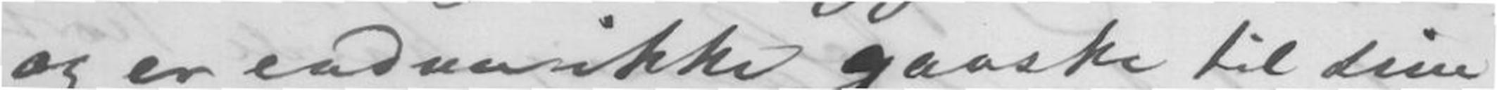og er endnu ikke ganske til sine
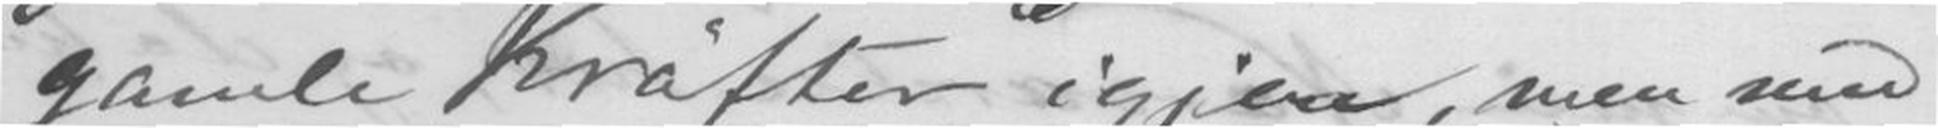gamle Kræfter igjen, men med
